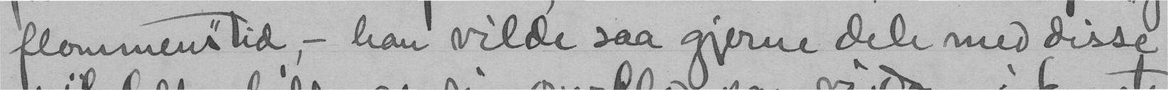flommens" tid, - han vilde saa gjerne dele med disse
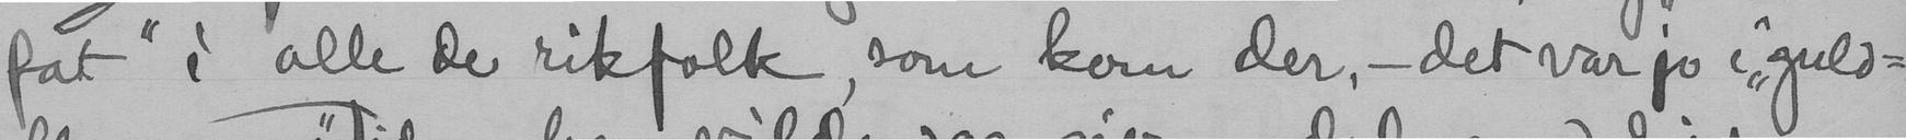fat" i alle de rikfolk, som kom der, - det var jo i "guld-
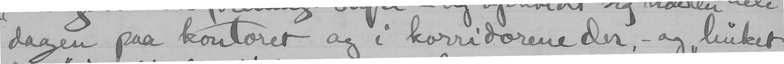dagen paa kontoret og i korridorene der, - og "huket
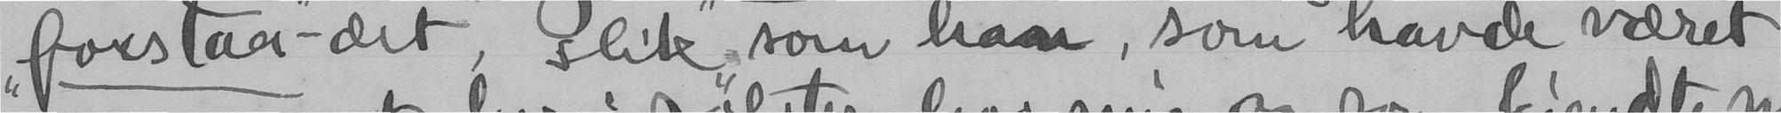"forstaa" - det, slik" som han, som havde været
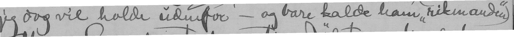jeg dog vil holde udenfor - og bare kalde ham "rikmanden")
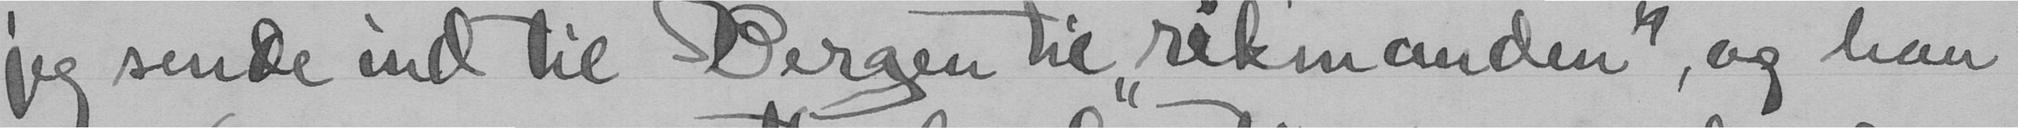jeg sende ind til Bergen til "rikmanden", og han
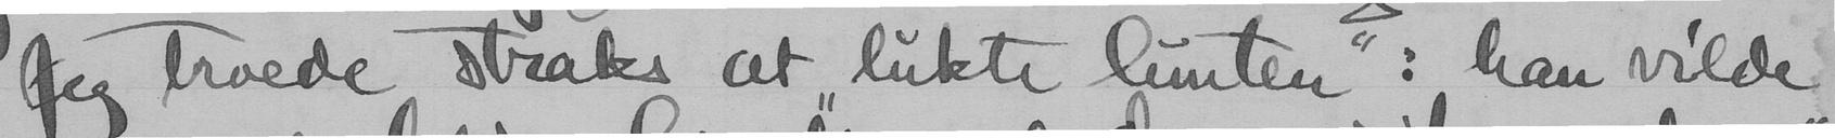Jeg troede straks at "lukte lunten": han vilde
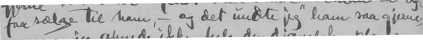faa sælge til ham, - og det undte jeg ham saa gjerne;
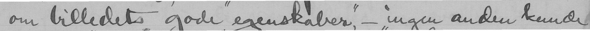om billedets gode egenskaber", - "ingen anden kunde
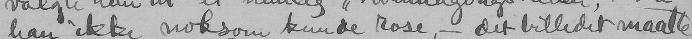han ikke noksom kunde rose, - det billedet maatte
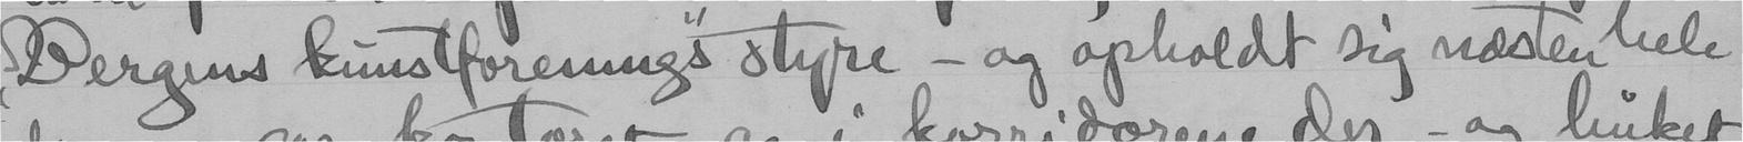"Bergens kunstforenings" styre - og opholdt sig næsten hele
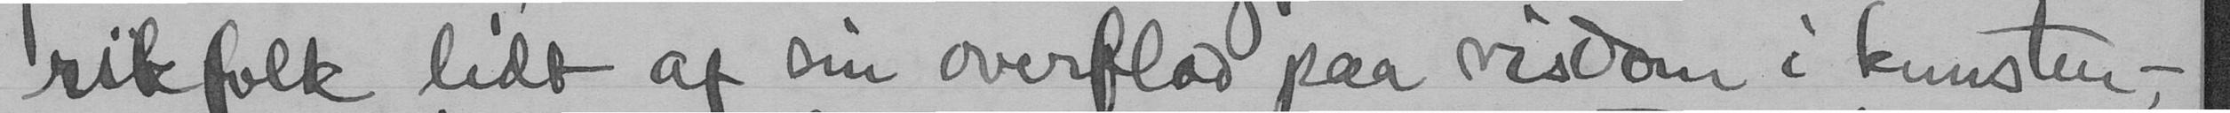rikfolk lidt af sin overflod paa visdom i kunsten,-
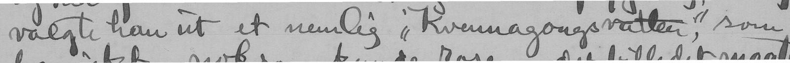vælgte han ut et nemlig "Kvennagongsvatten", som
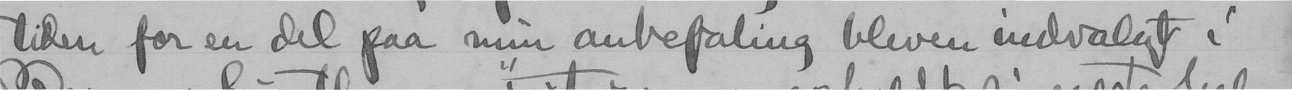tiden for en del paa min anbefaling bleven udvalgt i
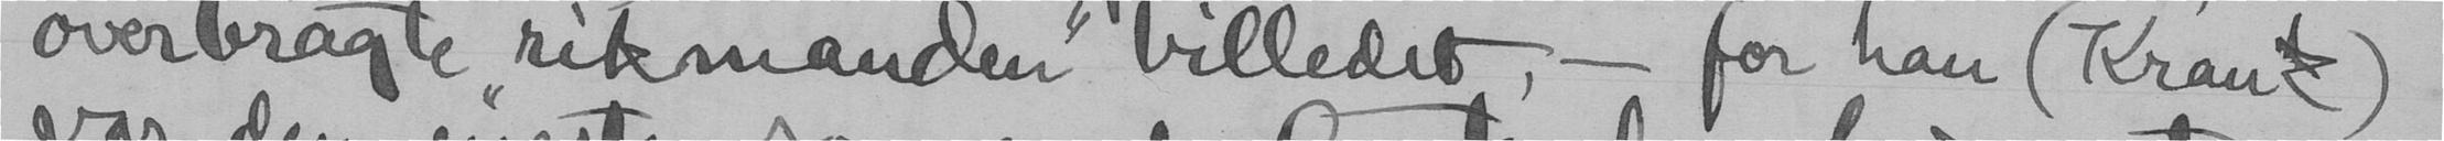overbragte "rikmanden" billedet - for han (Kranz)
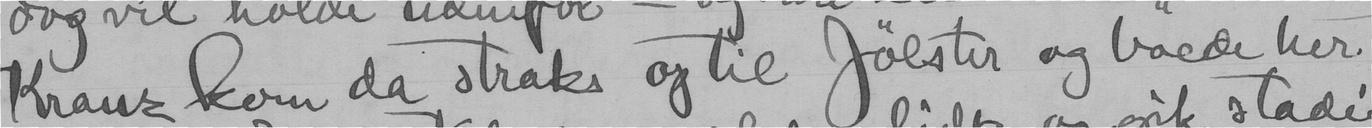Kranz kom da straks op til Jølster og boede her
